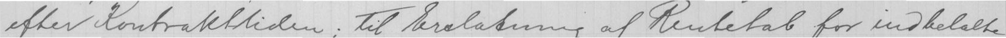efter Kontraktiden; til Erstatning af Rentetab for indbetalte
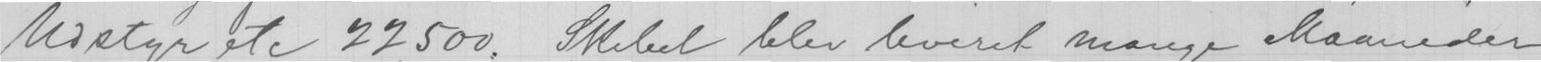Udstyr etc 22,500. Skibet blev leveret mange Maaneder
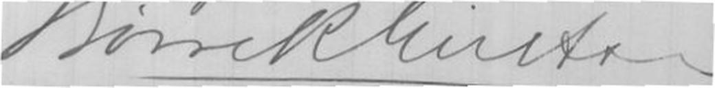Børre R Giertsen
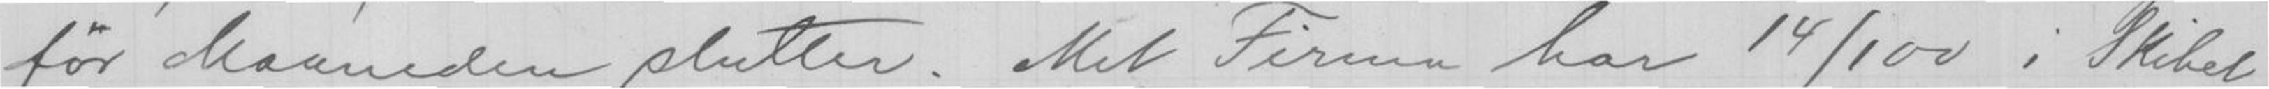før Maaneden slutter. Mit Firma har 14/100 i Skibet
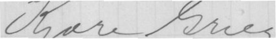Kjære Grieg
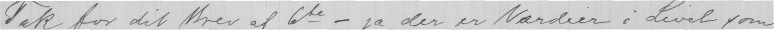Tak for dit Brev af 6te - ja der er Værdier i Livet som
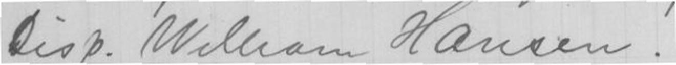Disp. William Hansen.
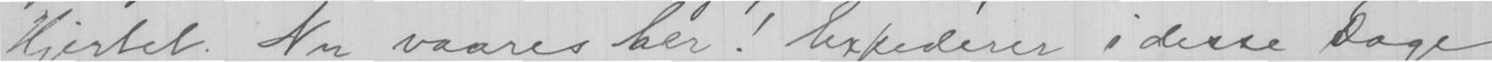Hjertet. Nu vaares her! Expederer i disse Dage
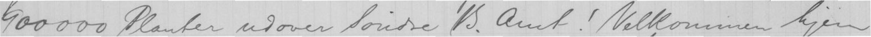900,000 Planter udover Søndre B. Amt! Velkommen hjem
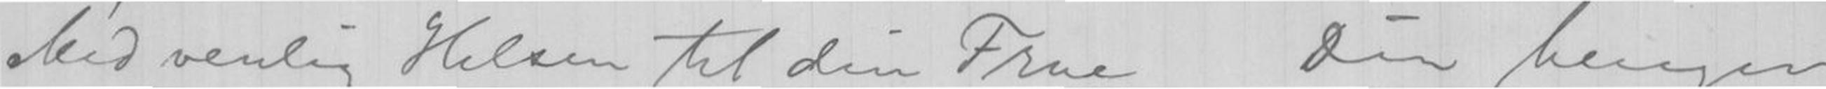Med venlig Hilsen til din Frue Din hengivne
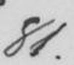81.
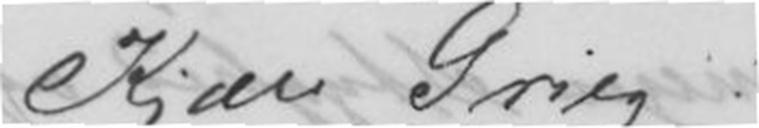Kjære Grieg!
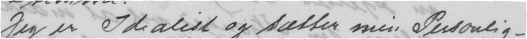Jeg er Idealist og sætter min Personlig-
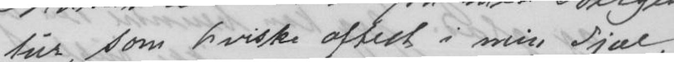tur, som hviske oftest i min Sjæl,
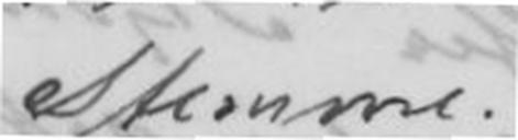Stemme.
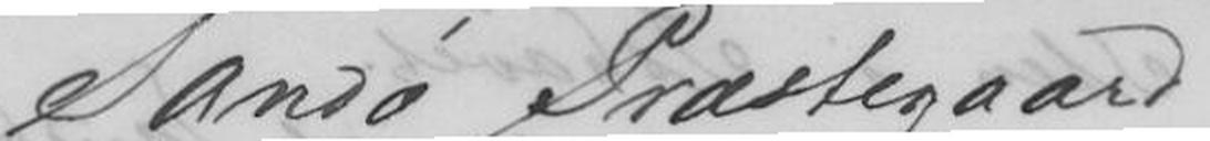Sandø Præstegaard
